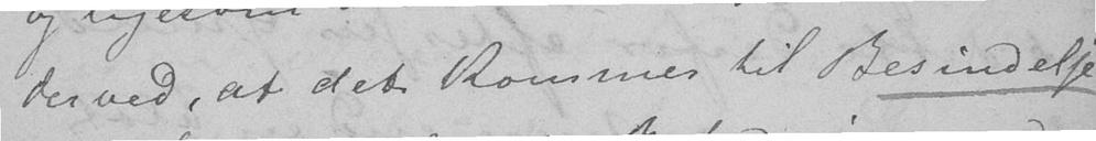derved, at det kommer til Besindelse
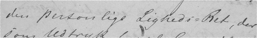den personlige Ligheds-Ret, der
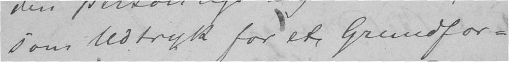som Udtryk for et Grundfor-
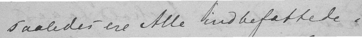saaledes ere Alle indbefattede i
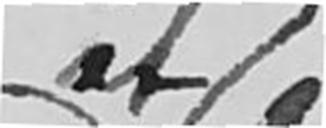et
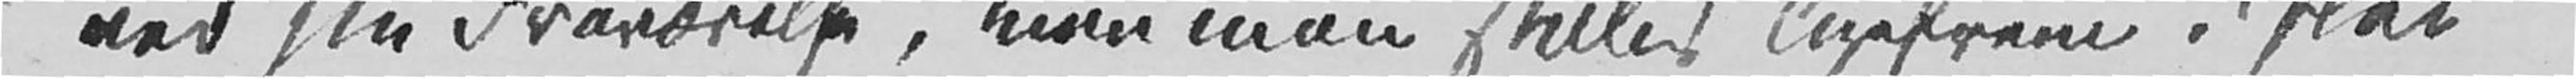ved sin Fraværelse, men man stilles ligefrem i slet
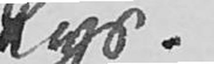Lys.
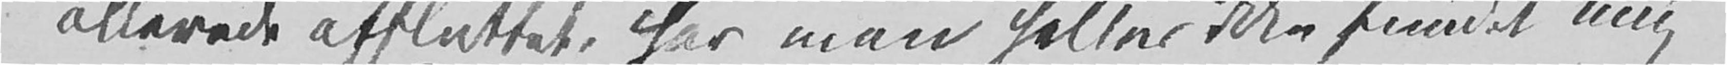allerede afsluttet, har man heller ikke fundet mig
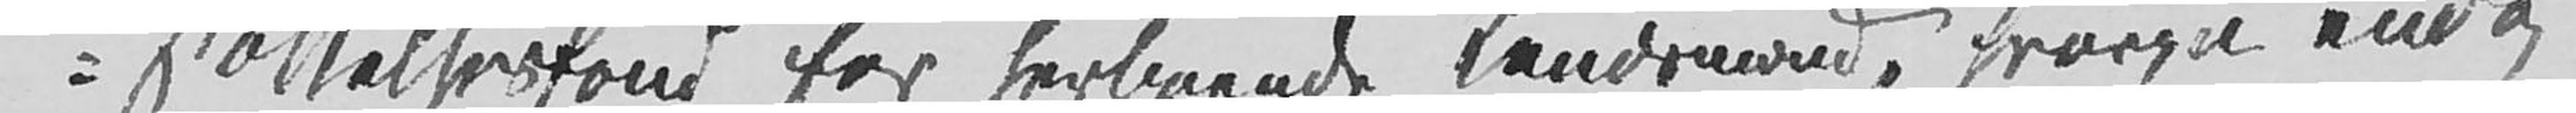støttelsesfond for herboende Landsmænd, hvorpå endog
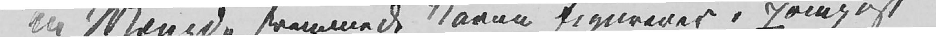en Mængde fremmede Navne figurerer i pompøst
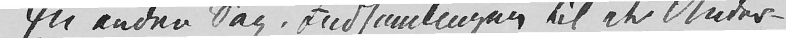En anden Sag. Indsamlingen til et Under¬
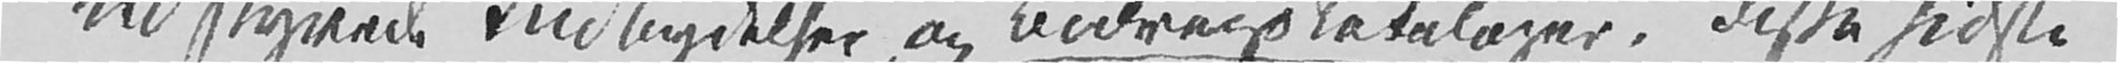udstyrede Indbydelser og Bidragskataloger, disse sidste
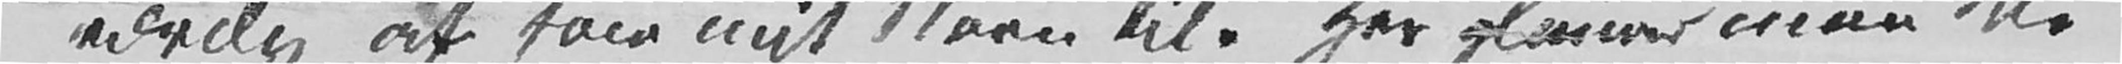værdig at føie mit Navn til. Her glimrer man ikke
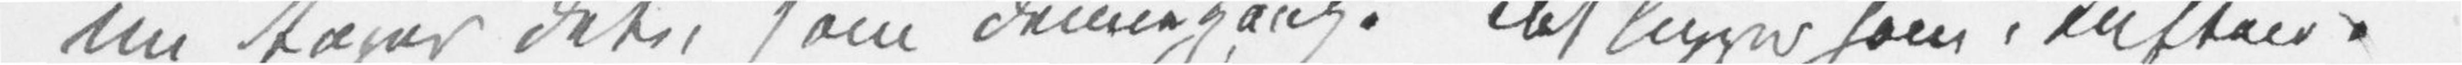nu tager det, som dennegang. Det ligger som i Luften.
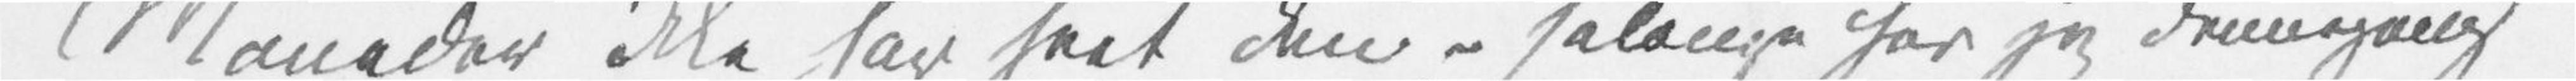Måneder ikke har seet den – sålænge har jeg dennegang
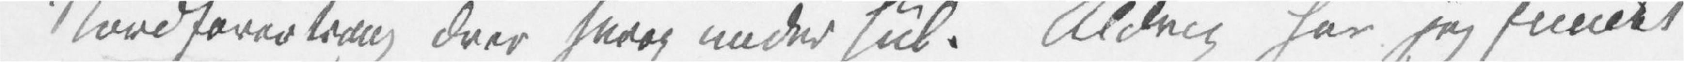Nordfarertrang drev herop under Jul. Aldrig har jeg fundet
